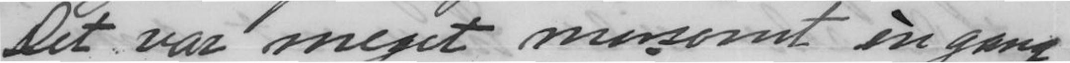Det var meget morsomt èn gang
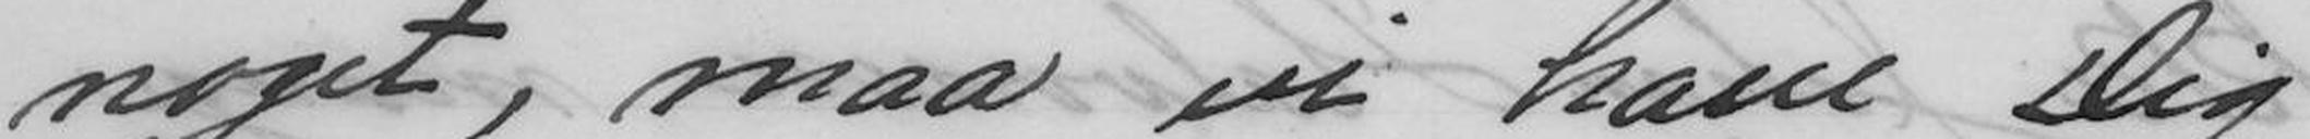noget, maa vi have Dig
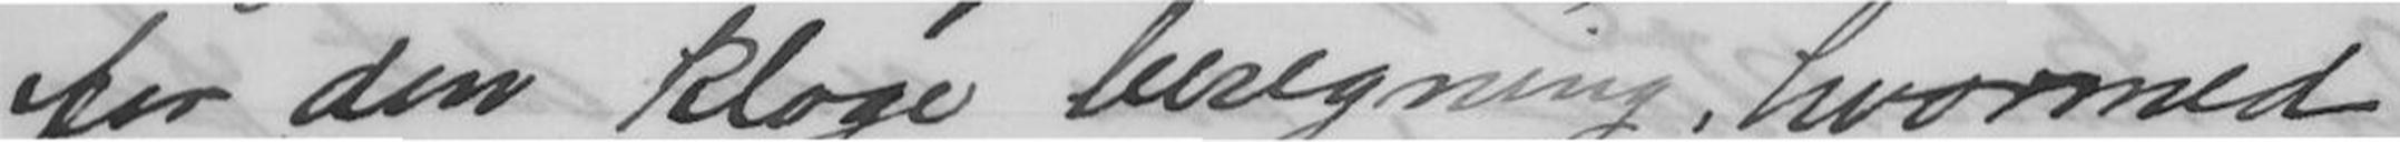for den kloge beregning, hvormed
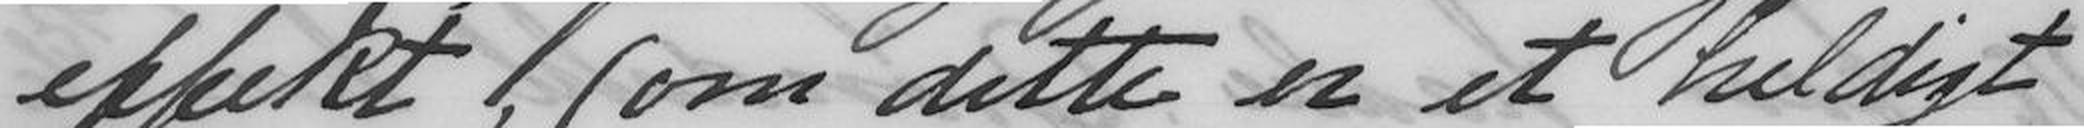effekt, (om dette er et heldigt
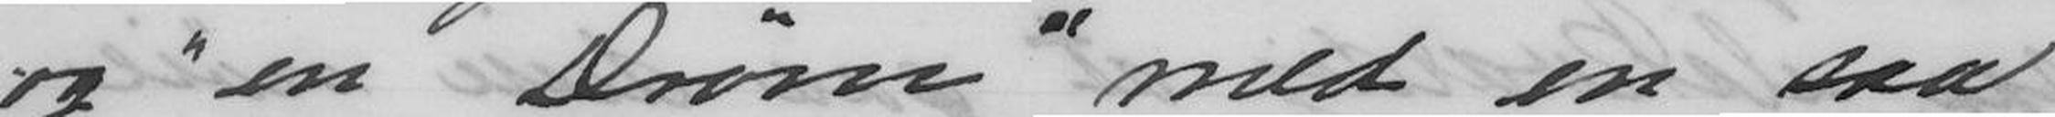og "en Drøm" med en saa
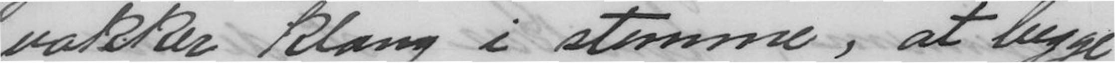vakker klang i stemme, at begge
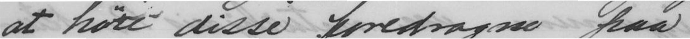at høre disse foredragne paa
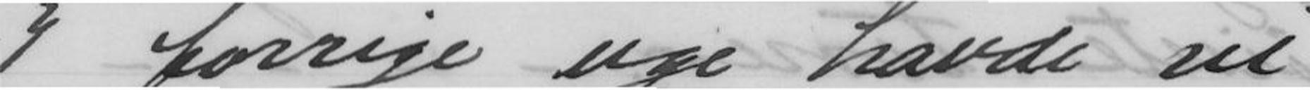I forrige uge havde vi
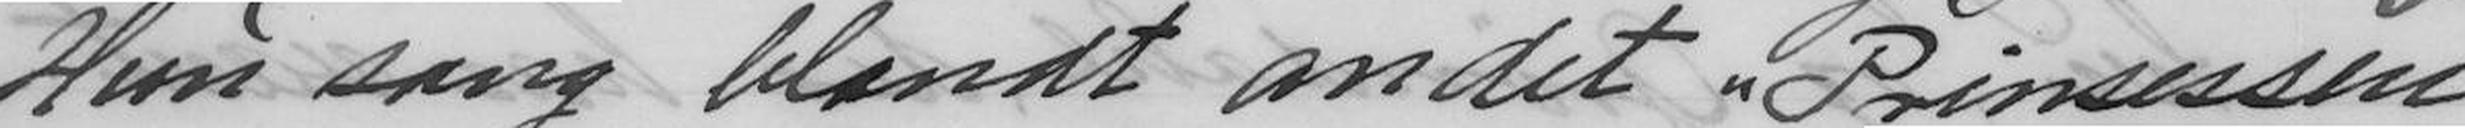Hun sang blandt andet "Prinsessen
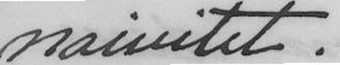naivitet.
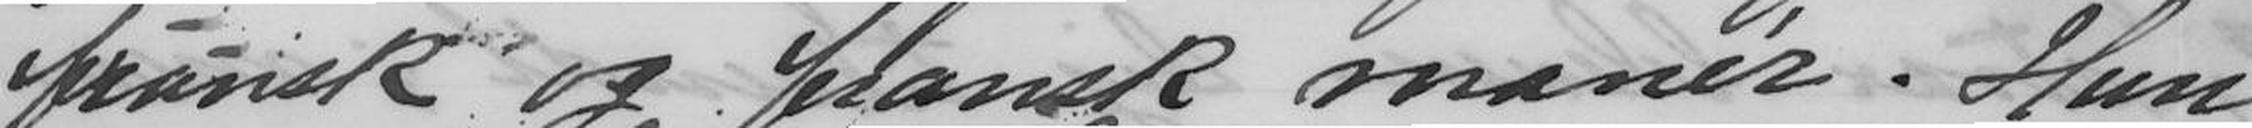fransk og fransk manér. Hun
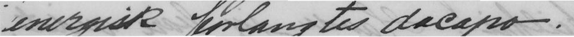energisk forlangtes dacapo.
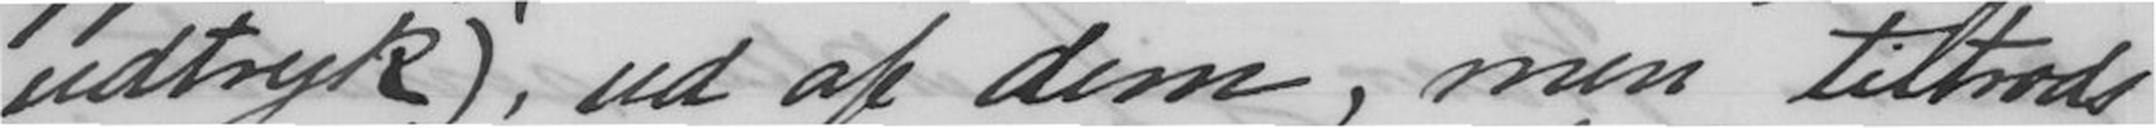udtryk), ud af dem, men tiltrods
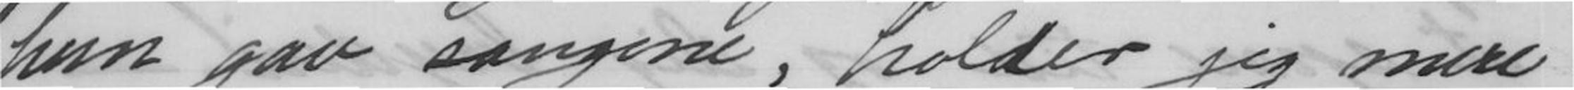hun gav sangene, holder jeg mere
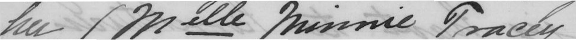her (Melle Minnie Tracey
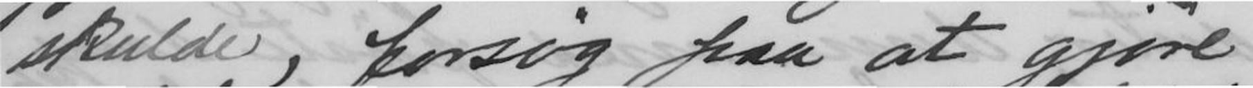skulde, forsøg paa at gjøre
